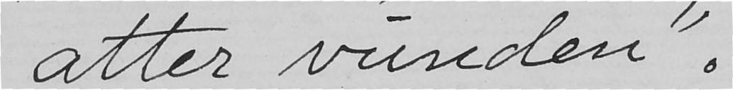atter vunden".
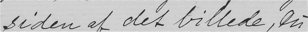siden af det billede, Du
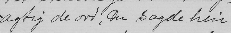agtig de ord, Du sagde hin
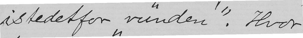istedetfor "vunden". Hvor
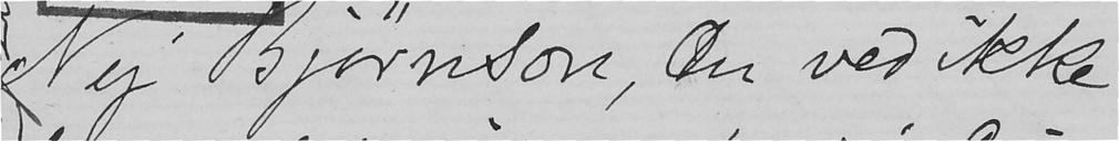Nej Bjørnson, Du ved ikke
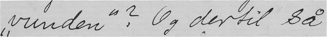"vunden"? Og dertil så
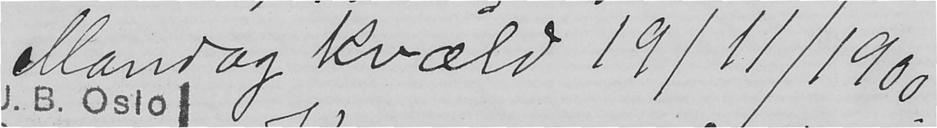Mandag kvæld 19/11/1900.
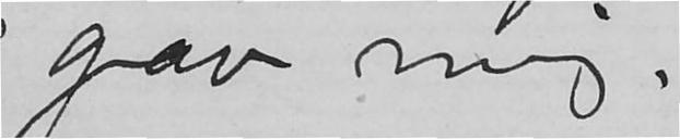gav mig.
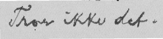Tror ikke det.
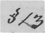§ 13
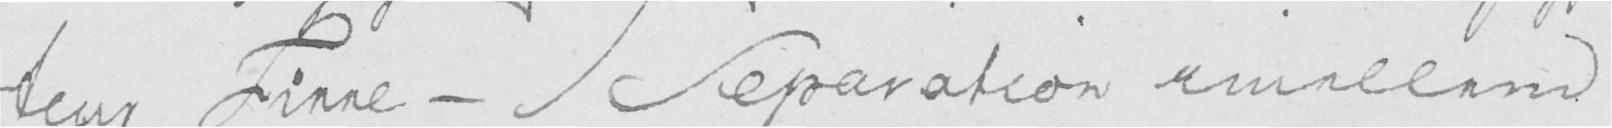teur Finne - Separation imellem
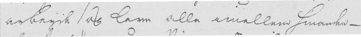arbeyde og leve alle imellem hinanden -
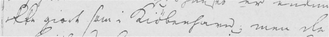ikke giort som i Kiøbenhavn; men de
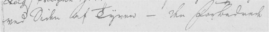ved Siden af Tyven - Den forbedrede
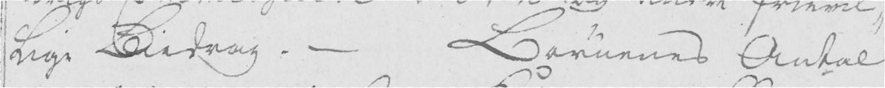lige bidrag. - Børnenes Antal
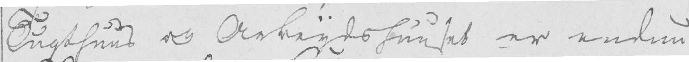Tugthuus og Arbeydshuuset er endnu
